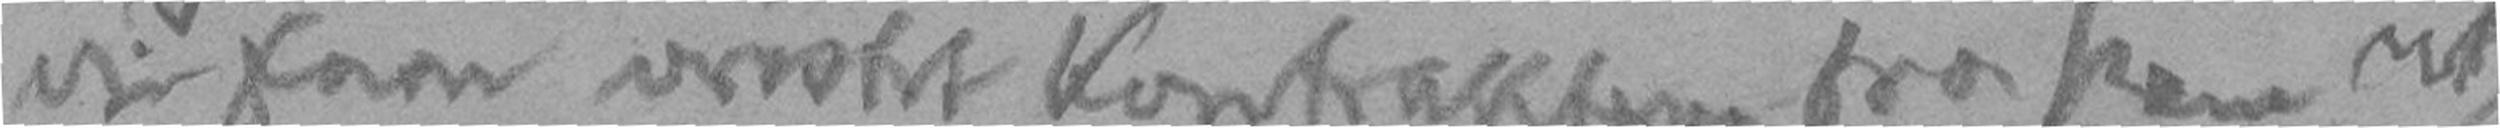vi faar vristet Kontrakten fra ham ut
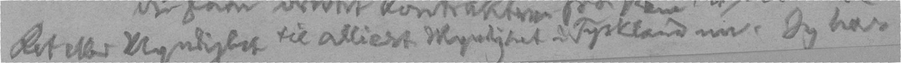Ret eller Myndighet til alliert Myndighet i Tyskland nu. Jeg har
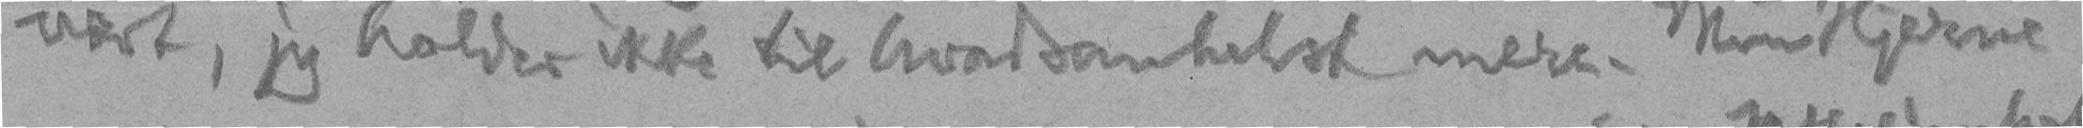vært, jeg holder ikke til hvadsomhelst mere. Min Hjerne
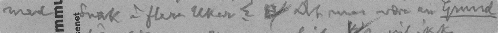med Snak i flere Uker? 3) Det maa være en Grund
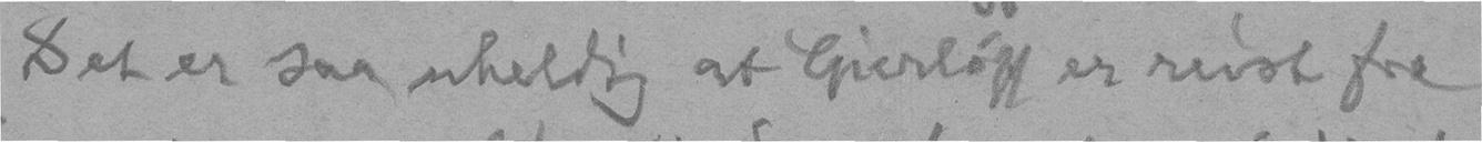Det er saa uheldig at Gierløff er reist fra
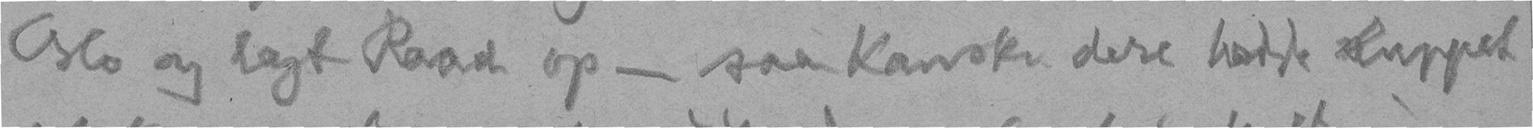Oslo og lagt Raad op - saa kanske dere hadde sluppet
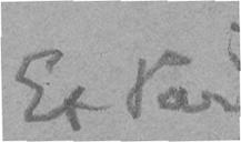Et Par
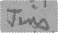Ting
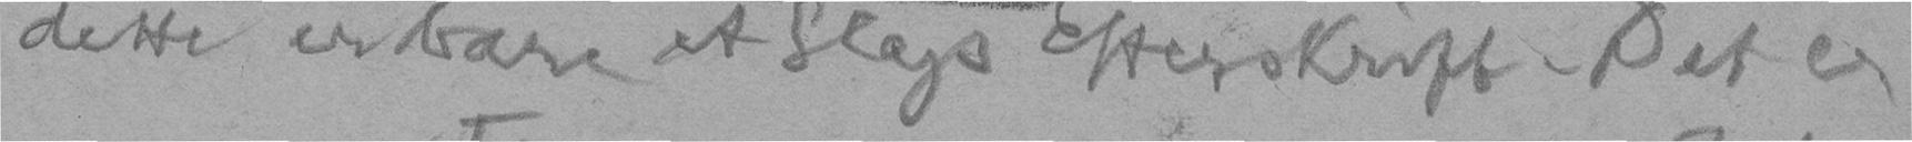dette er bare et Slags Efterskrift. Det er
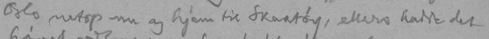Oslo netop nu og hjem til Skaatøy, ellers hadde det
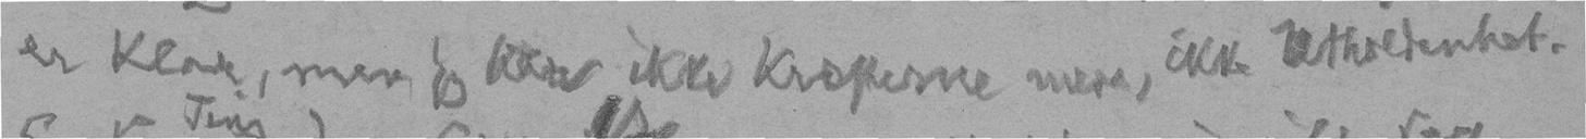er klar, men jeg har ikke Kræfterne mere, ikke Utholdenhet.
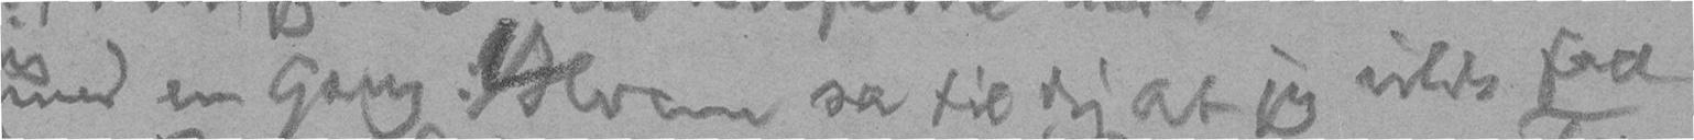med en Gang. 1) Hvem sa til dig at jeg vilde faa
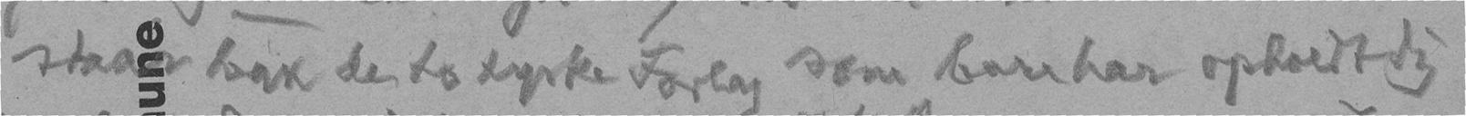staar bak de to tyske Forlag som bare har opholdt dig
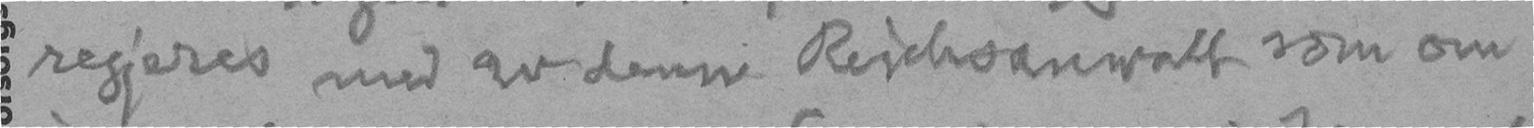regjeres med av denne Reichsanwalt som om
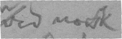ved norsk
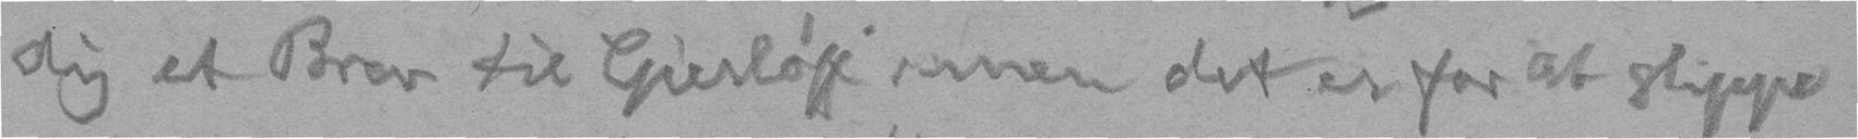dig et Brev til Gierløff men det er for at slippe
